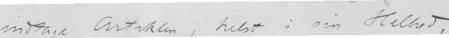indtage Artiklen, helst i sin Helhed,
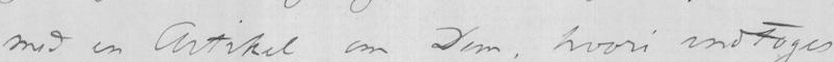med en Artikel om Dem, hvori indtages
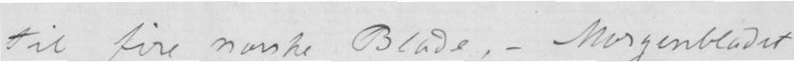til fire norske Blade, - Morgenbladet,
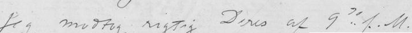Jeg modtog rigtig Deres af 9de f.M.
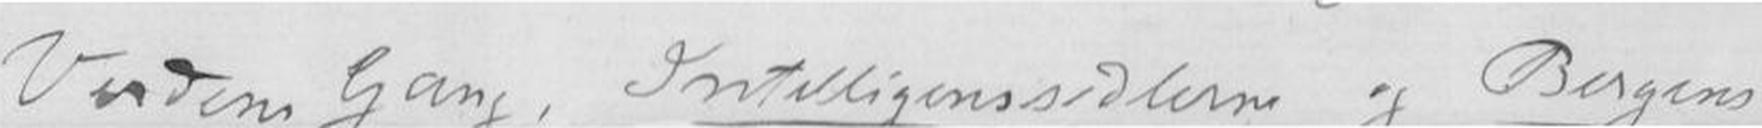Verdens Gang, Intelligenssedlerne og Bergens
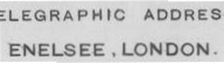ENELSEE.LONDON
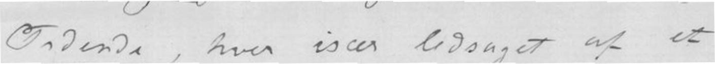Tidende, hver især ledsaget af et
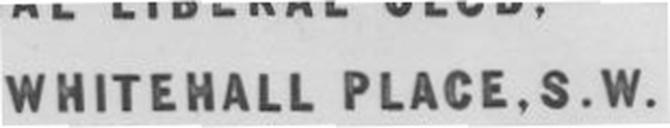WHITEHALL PLACE,S.W.
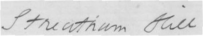Streatham Hill.
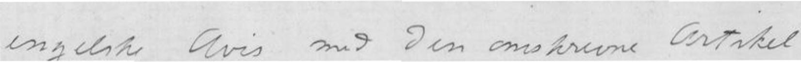engelske Avis med den omskrevne Artikel
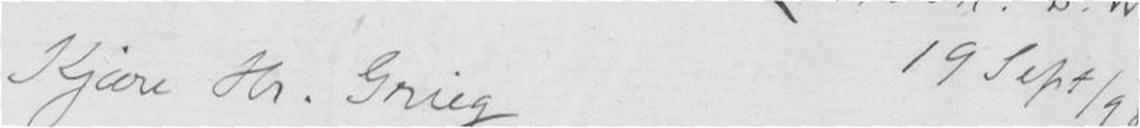19 Sept/98
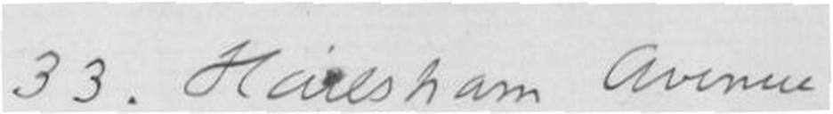33. Hailsham Avenue
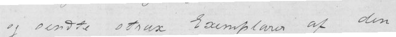og sendte strax Exemplarer af den
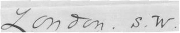London. S.W.
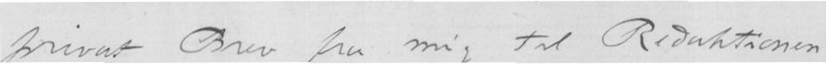privat Brev fra mig til Redaktionen
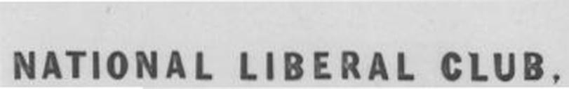NATIONAL LIBERAL CLUB.
